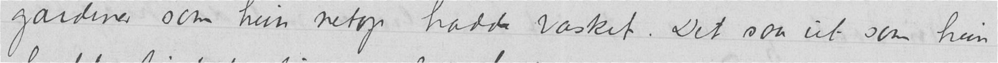gardiner som hun netop hadde vasket. Det saa ut som hun
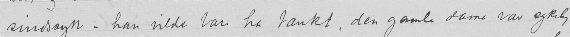sindssyk – han vilde bare ha tænkt, den gamle dame var sykelig
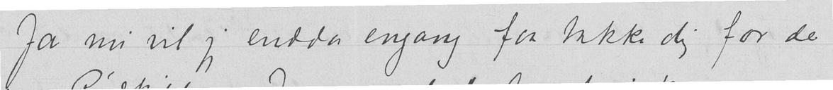Ja nu vil jeg endda engang faa takke dig for de
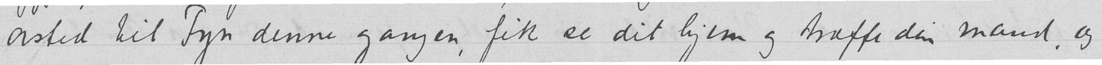avsted til Fyn denne gangen, fik se dit hjem og træffe din mand, og
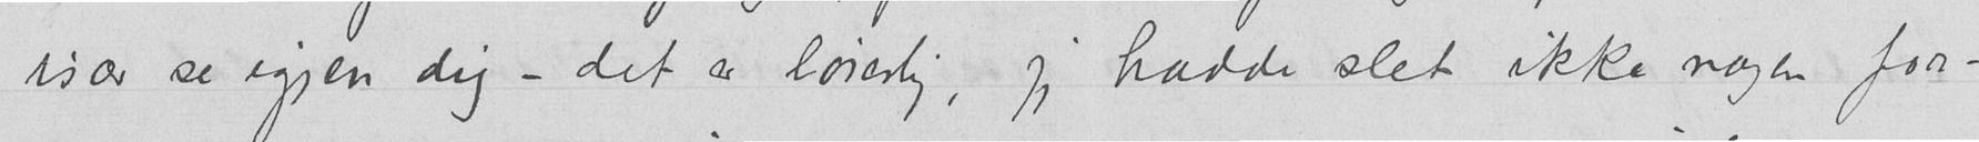især se igjen dig – det er løierlig, jeg hadde slet ikke nogen for-
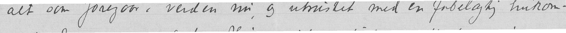alt som foregaar i verden nu, og utrustet med en fabelagtig hukom-
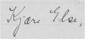Kjære Else,
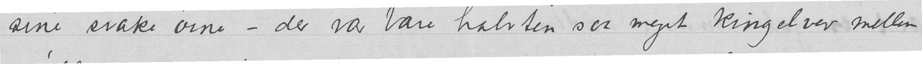sine svake øine – der var bare halvten saa meget kingelvev mellem
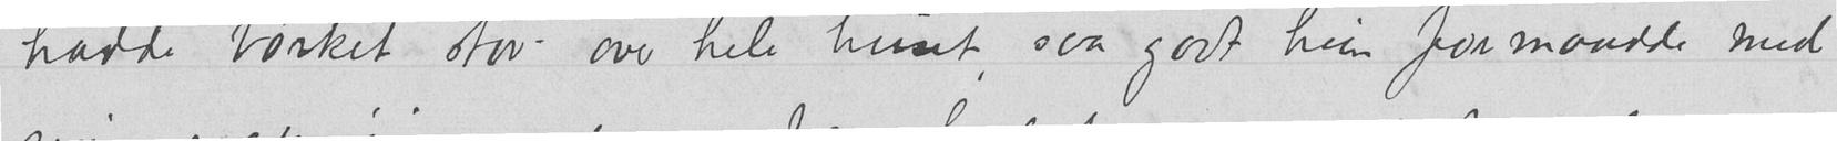hadde tørket støv – over hele huset, saa godt hun formaadde med
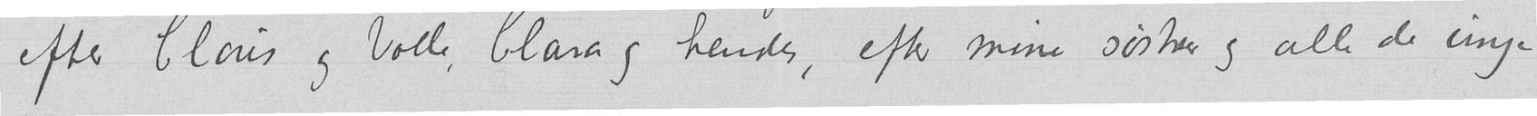efter Clous og Volle, Clara og hendes, efter mine søstrer og alle de unge
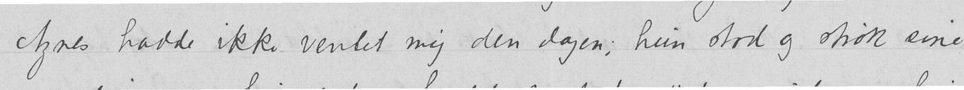Agnes hadde ikke ventet mig den dagen; hun stod og strøk sine
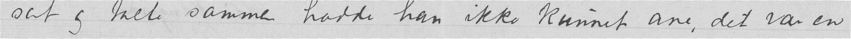sat og talte sammen hadde han ikke kunnet ane, det var en
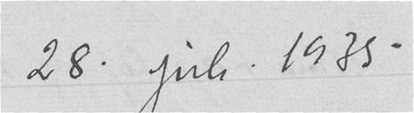28. juli. 1935.
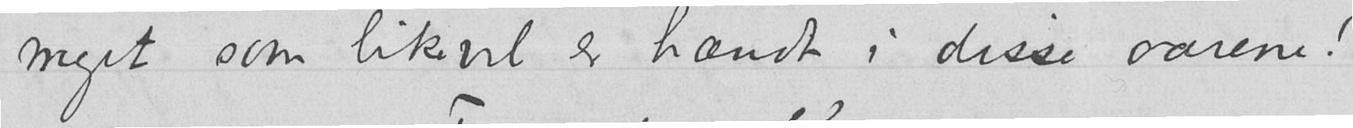meget som likevel er hændt i disse aarene!
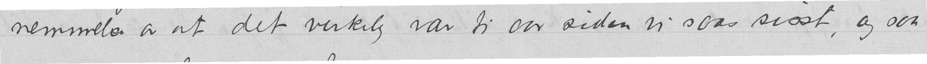nemmelse av at det virkelig var ti aar siden vi saas sisst, og saa
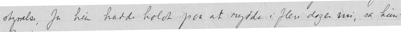styrrelse, for hun hadde holdt paa at rydde i flere dager nu, sa hun.
